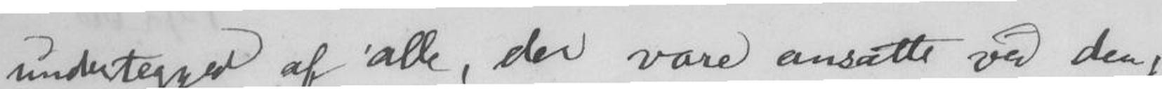undertegned af alle, der vare ansatte ved den,
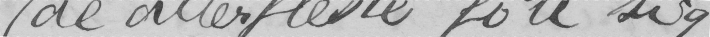de aller fleste føle sig
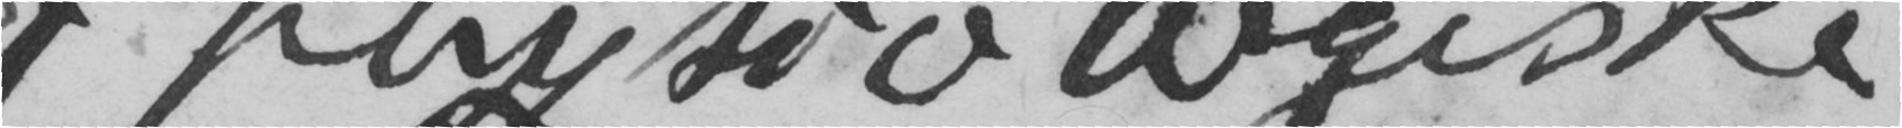physiologiske
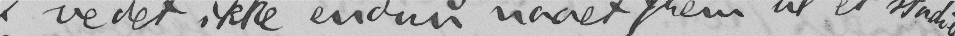vedet ikke endnu naaet frem til et stadium
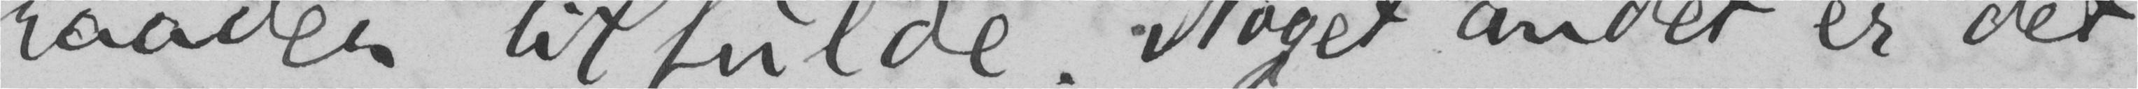raader tilfulde. Noget andet er det
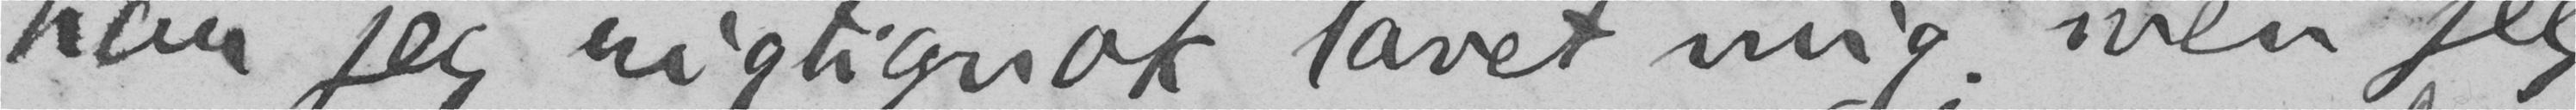har jeg rigtignok lavet mig; men jeg
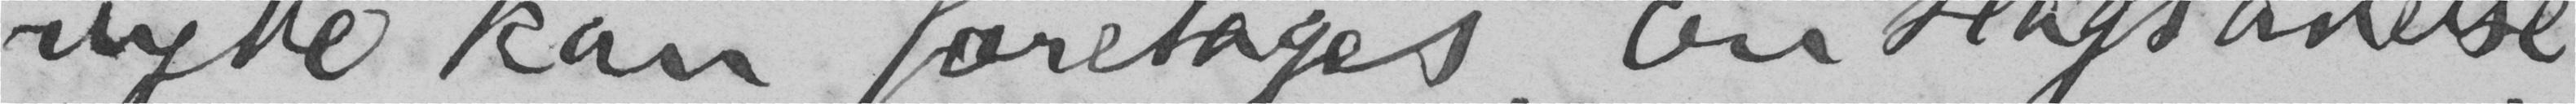nytte kan foretages. En slags anelse
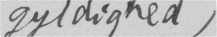gyldighed
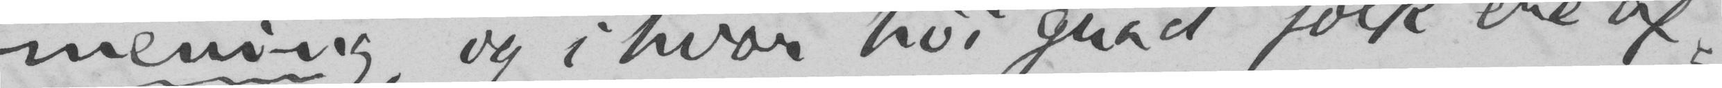mening, og i hvor høi grad folk ere af-
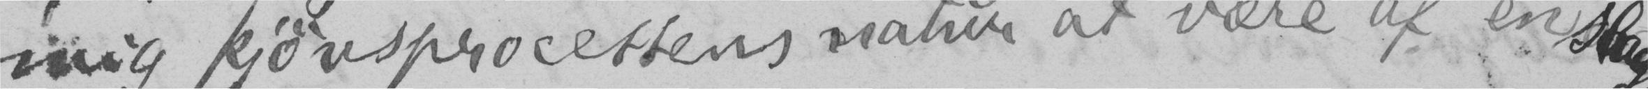mig kjønsprocessens natur at være af enslags
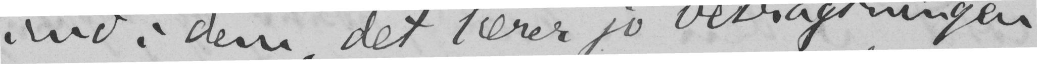ind i dem, det lærer jo betragtningen
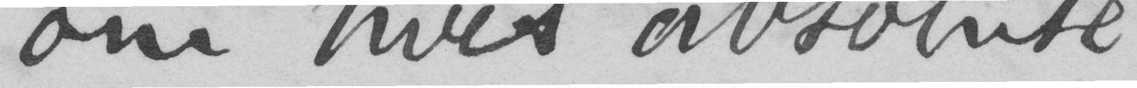om hvis absolute
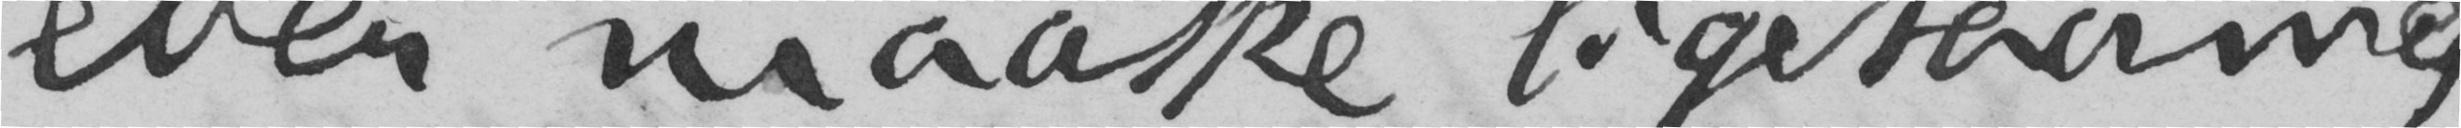eller maaske ligesaame
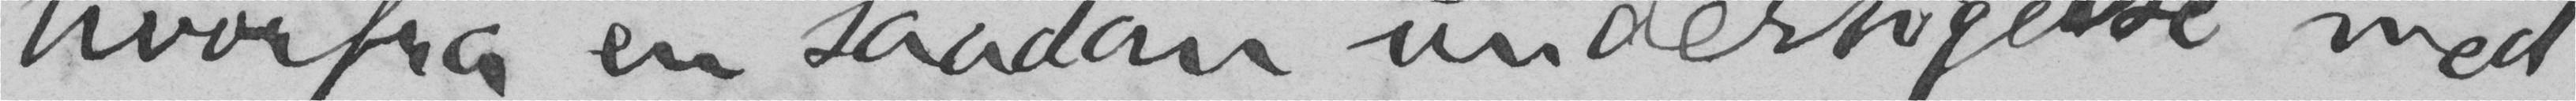hvorfra en saadan undersøgelse med
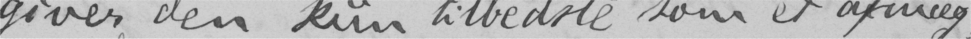giver den kun tilbedste som et afmæg-
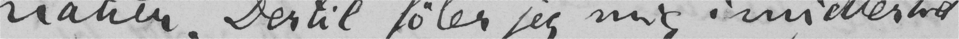natur. Dertil føler jeg mig imidlertid
